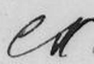er
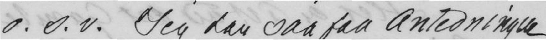o.s.v. Jeg har saa faa Anledninger
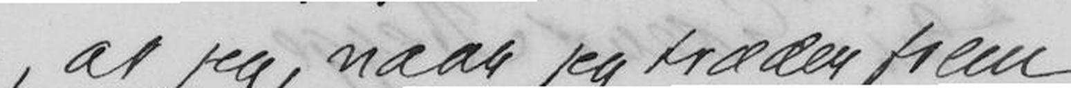, at jeg, naar jeg træder frem,
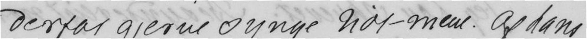Derfor gjerne synge hør-. Af hans
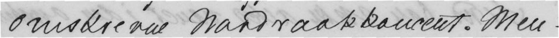omskrevne Nordraakkoncert. Men
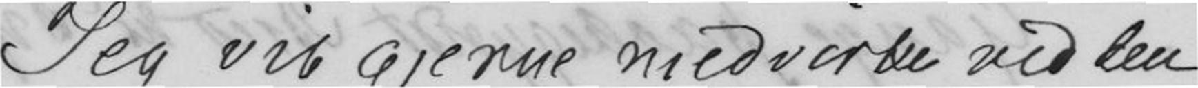Jeg vil gjerne medvirke ved den
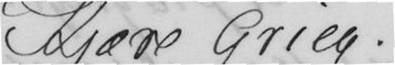Kjære Grieg
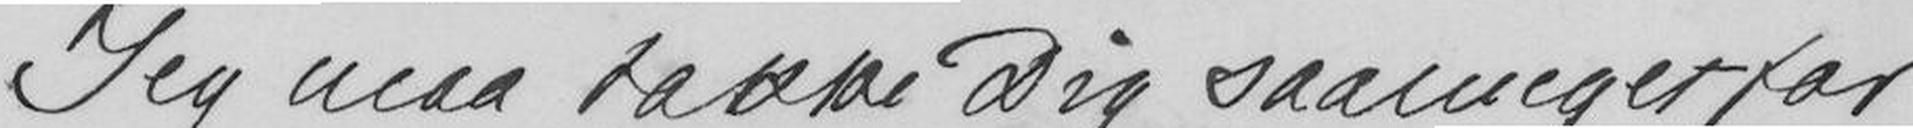Jeg maa takke Dig saameget for
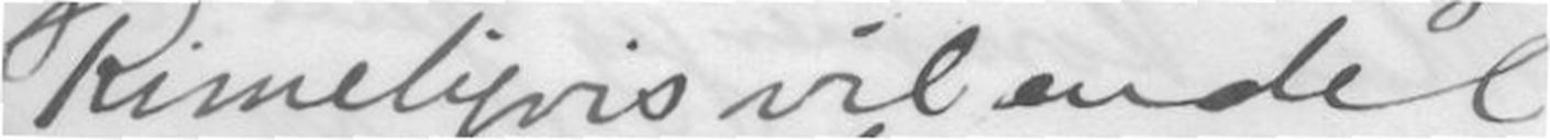Rimeligvis vil endel
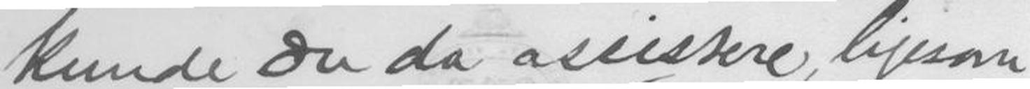kunde Du da assistere, ligesaa
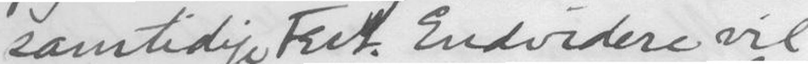samtidige Fest. Endvidere vil
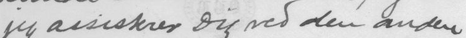jeg assisterer Dig ved den anden
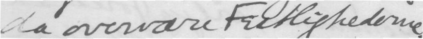da overvære Festlighederne.
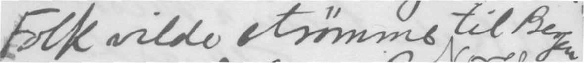Folk vilde strømme til Bergen
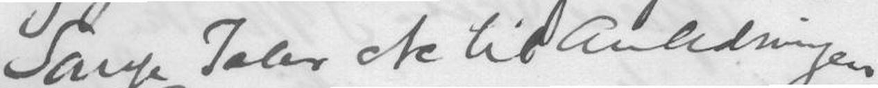Sange Taler etc til Anledningen.
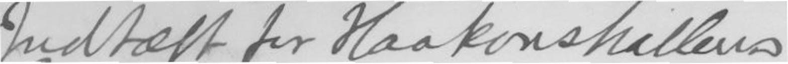Indtægt for Hakonshallens
